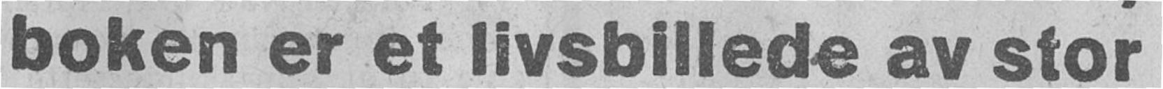boken er et livsbillede av stor
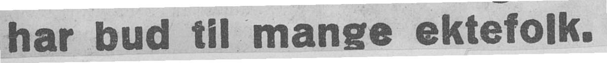har bud til mange ektefolk.
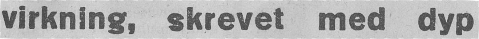virkning, skrevet med dyp
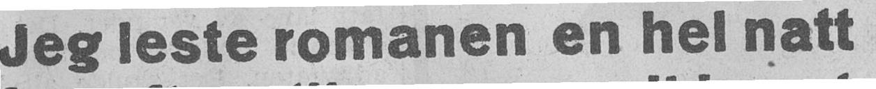Jeg leste romanen en hel natt
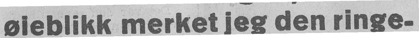øieblikk merket jeg den ringe-
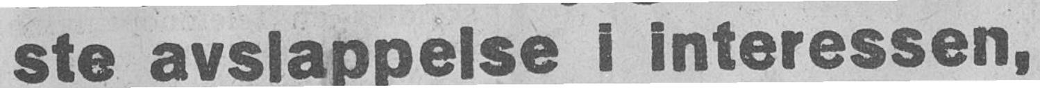ste avslappelse i interessen,
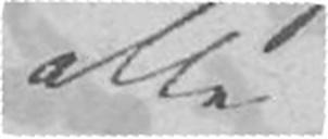alle
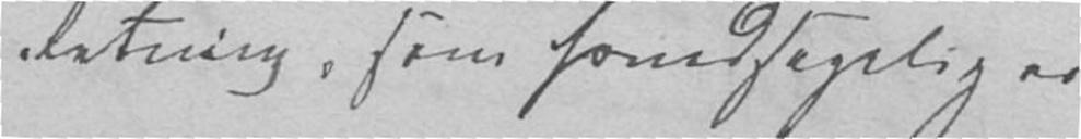Retning, som hovedsagelig er
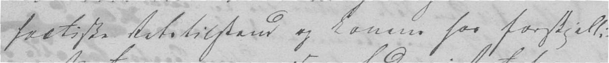factiske Retstilstand og Lovene for forskjælli-
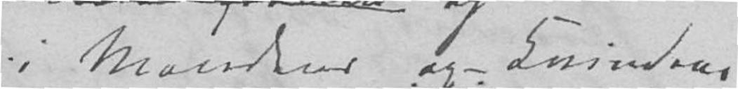i Mandens og Qvindens
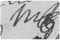Med
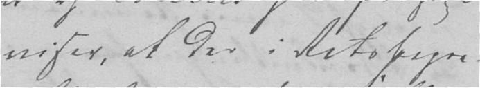viser, at der i Retsbegre-
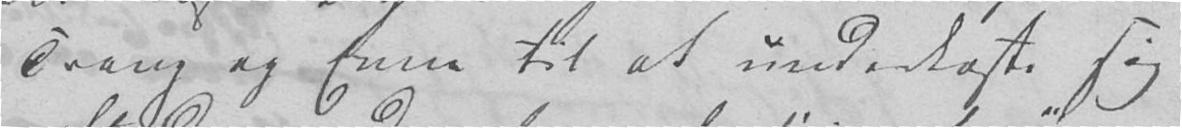Trang og Evne til at underkaste sig
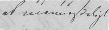et menneskeligt
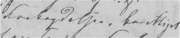Forbrydelser, berettiget
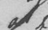at
